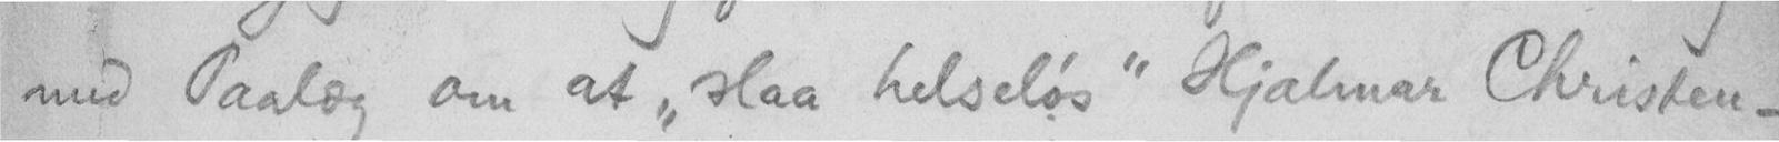med Paalæg om at "slaa helseløs" Hjalmar Christen-
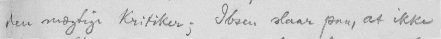den mægtige Kritiker; Ibsen slaar paa, at ikke
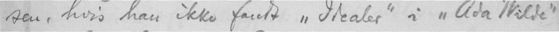sen, hvis han ikke fandt "Idealer" i "Ada Wilde"
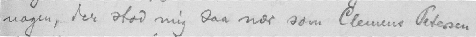nogen, der stod mig saa nær som Clemens Petersen
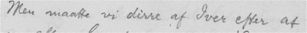Men maatte vi dirre af Iver efter at
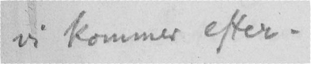vi kommer efter.
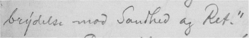brydelse mod Sandhed og Ret."
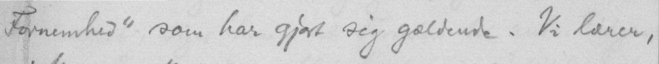Fornemhed" som har gjort sig gældende. Vi lærer,
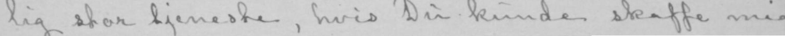lig stor tjeneste, hvis Du kunde skaffe mig
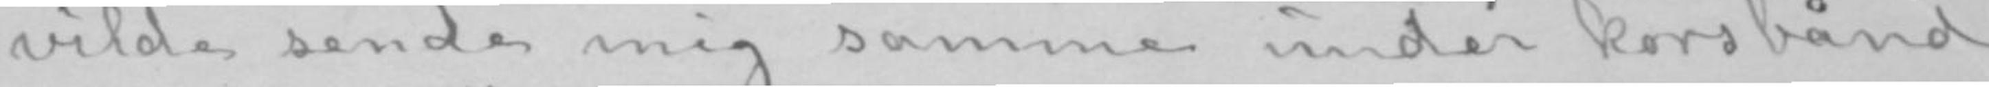vilde sende mig samme under korsbånd
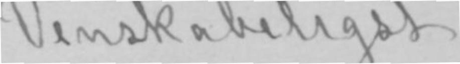Venskabeligst
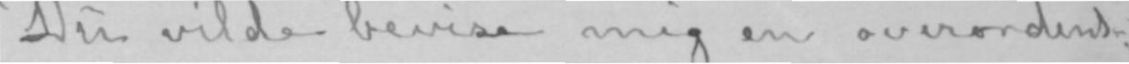Du vilde bevise mig en overordent-
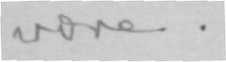være.-
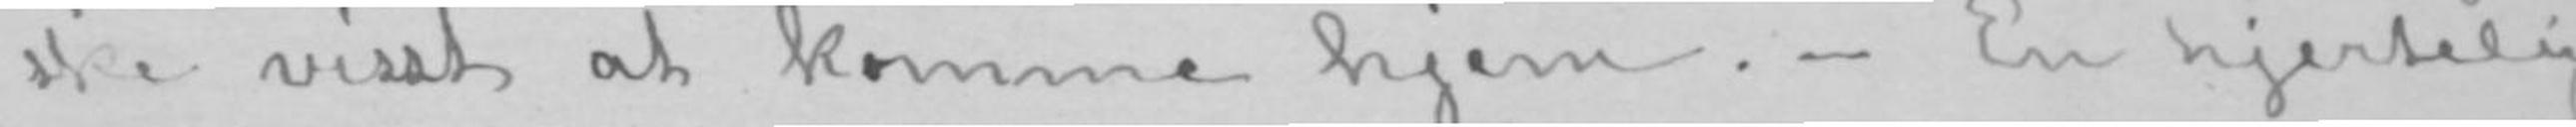ske visst at komme hjem.- En hjertelig
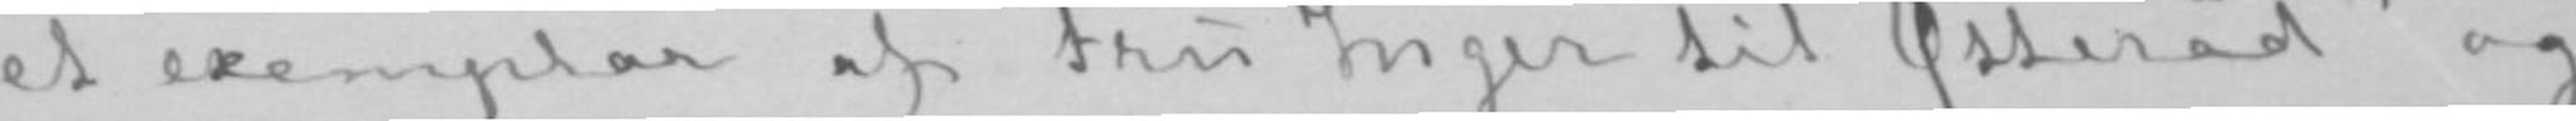NB! et exemplar af Fru Inger til Østeråd» og
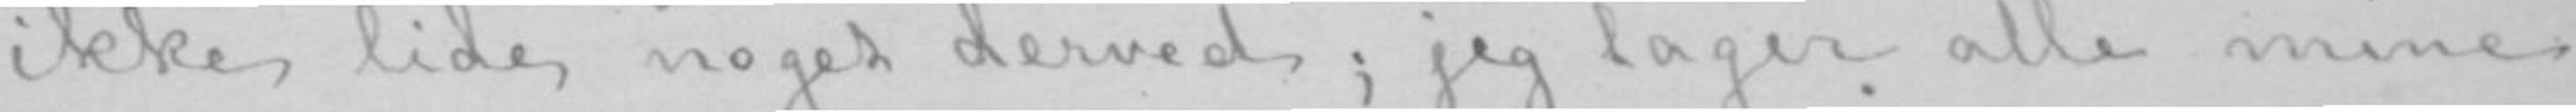ikke lide noget derved; jeg tager alle mine
